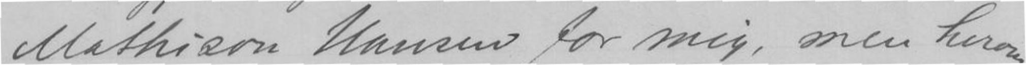Mathison Nansen for mig, men herom
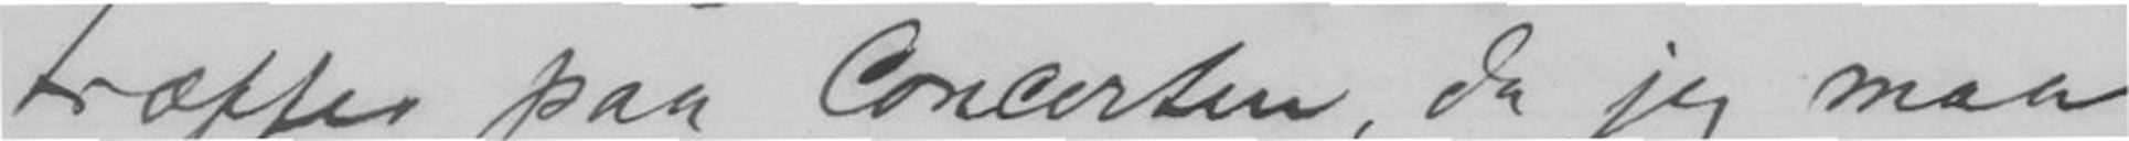træffes paa Concerten, da jeg maa
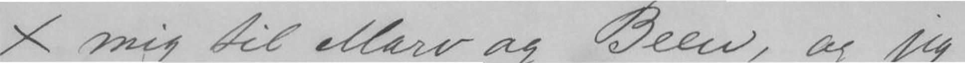mig til Marv og Been, og jeg
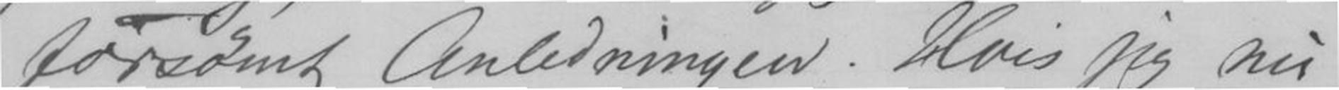forsømt Anledningen. Hvis jeg nu
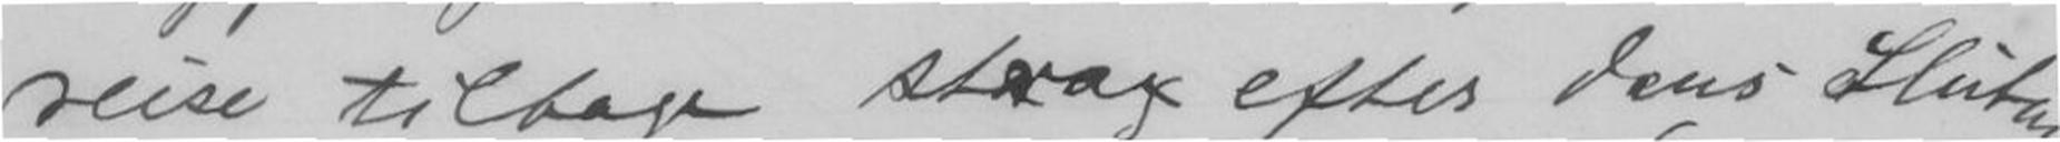reise tilbage strax efter dens Slutning.
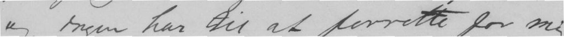og ingen har til at forrette for mig.
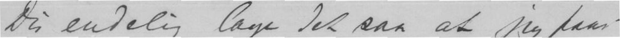Du endelig lage det saa at jeg faar
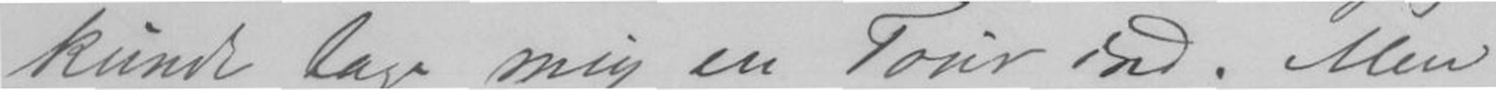kunde tage mig en Tour ind. Men
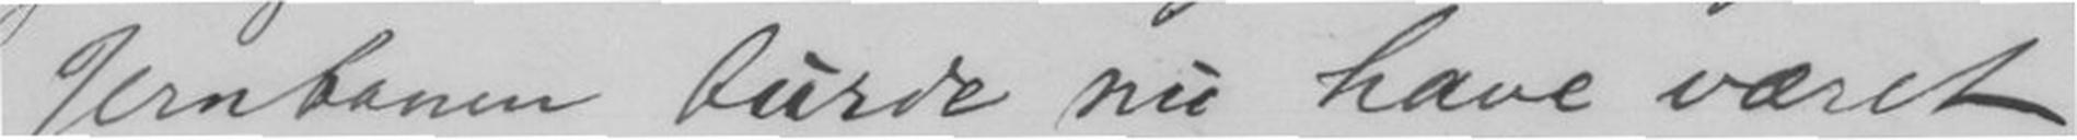Jernbanen burde nu have været
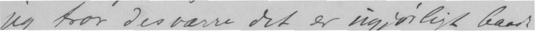jeg tror desværre det er ugjørligt baade
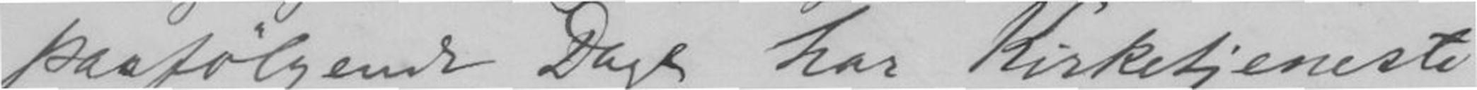paafølgende Dage har Kirketjeneste
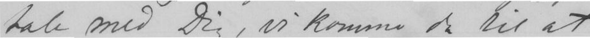tale med Dig, vi kommer da til at
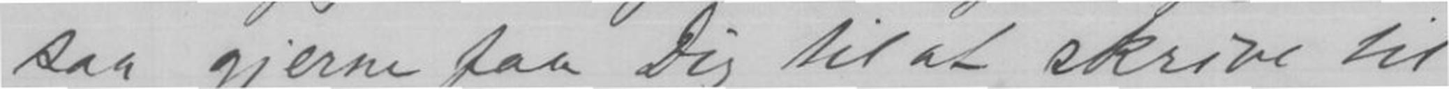saa gjerne faa Dig til at skrive til
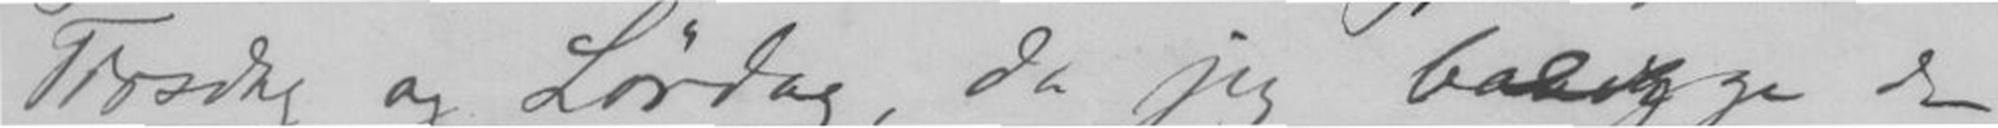Tirsdag og Lørdag, da jeg belægge de
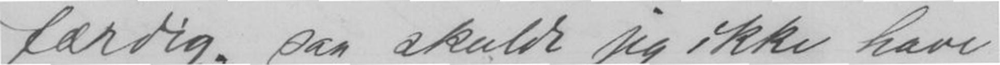færdig, saa skulde jeg ikke have
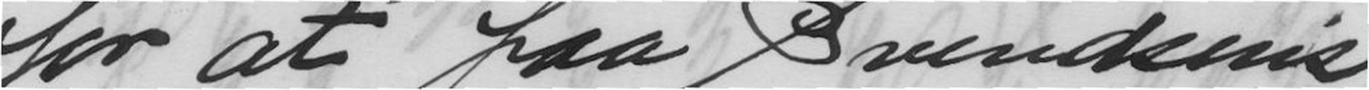for at faa Svendsens
